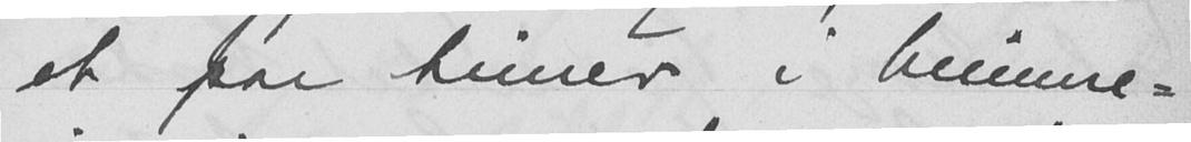et par timer i himme-
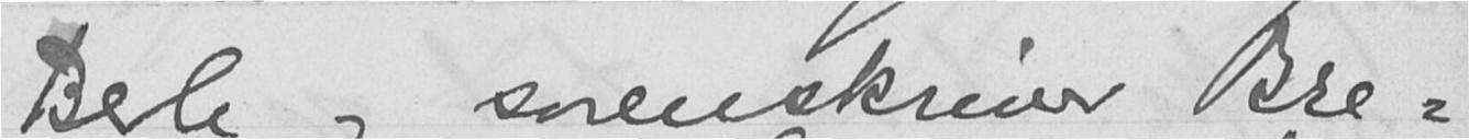Berle og sorenskriver Bre-
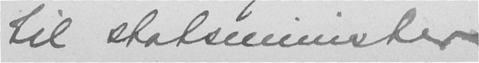til statsminster
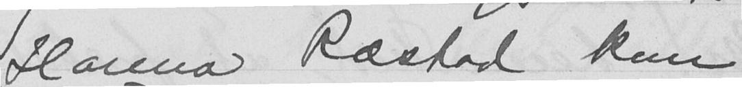Hanna Ræstad kom
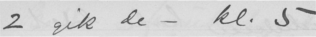2 gik de - kl. 5
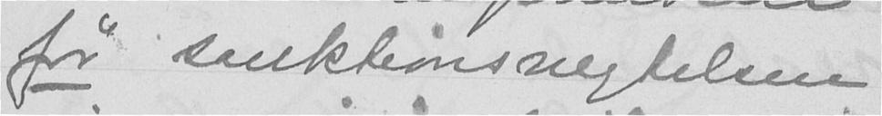før sanktionsnegtelsen
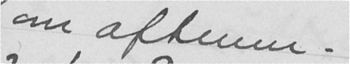om aftenen.
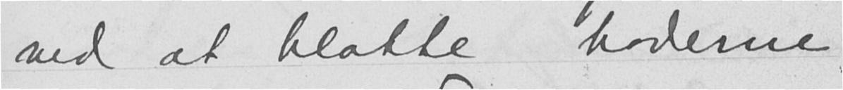ved at blotte hoderne
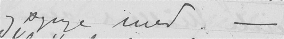og synge med. -
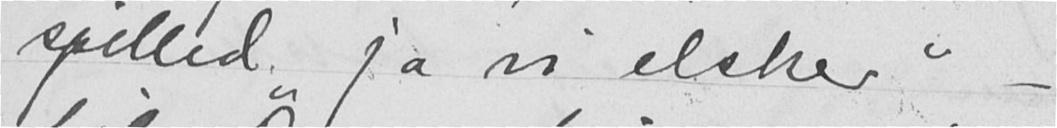spilled "ja vi elsker" -
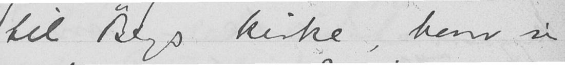til Bergs kirke, hvor vi
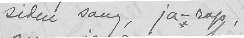siden sang, ja-rop,
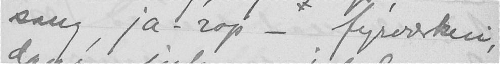sang, ja-rop - fyrværkeri,
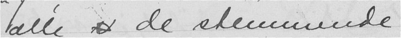alle e de stemmende
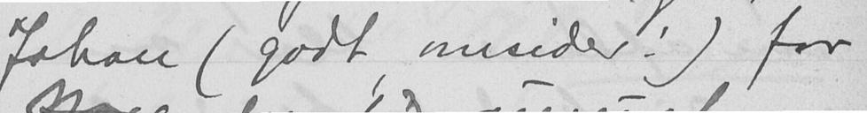Johan (godt omsider!) for
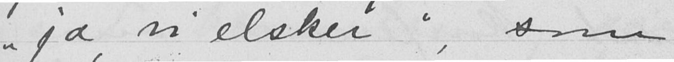"ja, vi elsker", som
